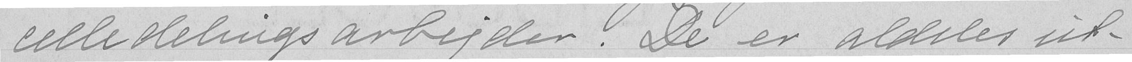celledelingsarbejder. De er aldeles ut-
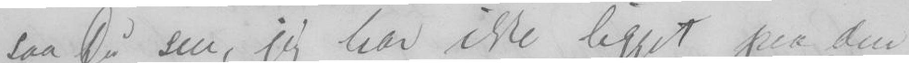saa Du ser, jeg har ikke ligget paa den
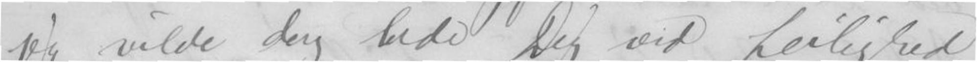jeg vilde dog bede Dig ved Leilighed
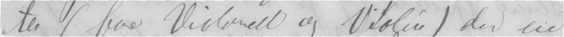ter (paa Violonell og Violin) der ere
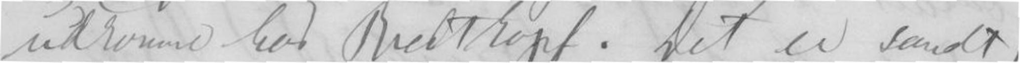udkomme hos Breitkopf. Det er sandt,
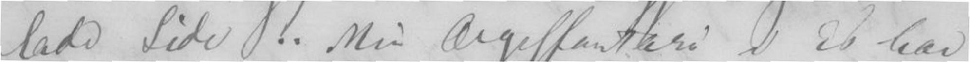lade Side.. Min Orgelfantasi i Es har
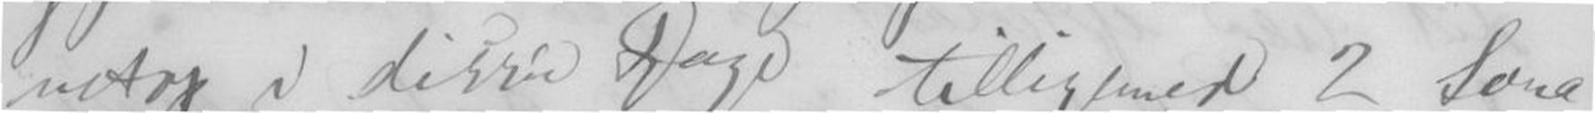netop i disse Dage tilligemed 2 Sona-
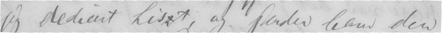jeg dedicert Liszt, og sender ham den
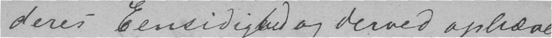deres Eensidighed og derved ophæve
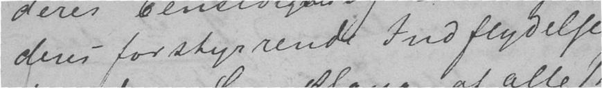deres forstyrrende Indflydelse
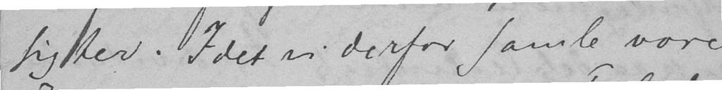sigter. I det vi derfor samle vore
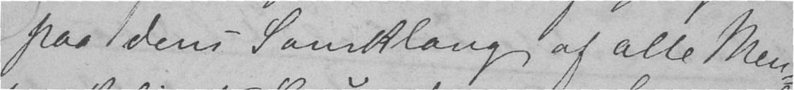paa dens Samklang af alle Men-
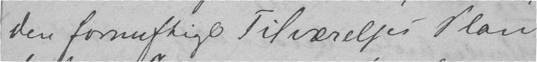den fornuftige Tilværelses Plan
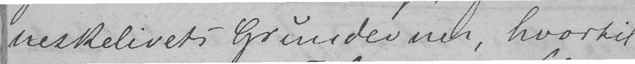neskelivets Grundevner, hvortil
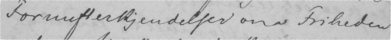Fornuftserkjendelser om Friheden
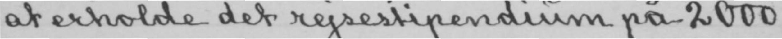at erholde det rejsestipendium på 2000
